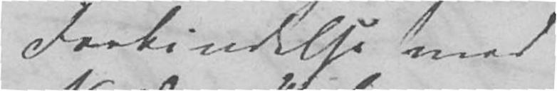Forbindelse med
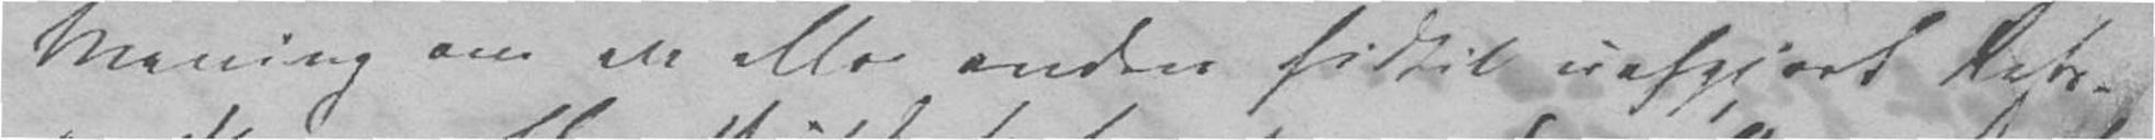Mening om en eller anden hidtil uafgjort Rets-
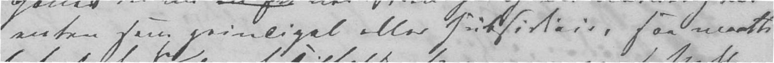enten som principal eller subsidiair, saa maatte
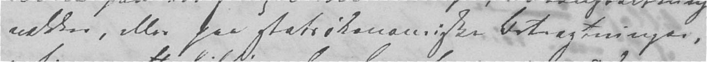vekker, eller paa statsøkonomiske Betragtninger,
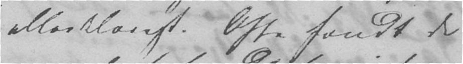allerklarest. Ofte fandt de
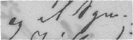og et Spm.
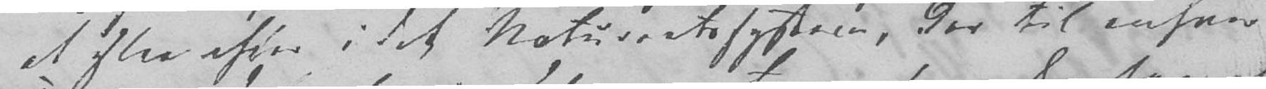at slaa efter i det Naturretssystem, der til enhver
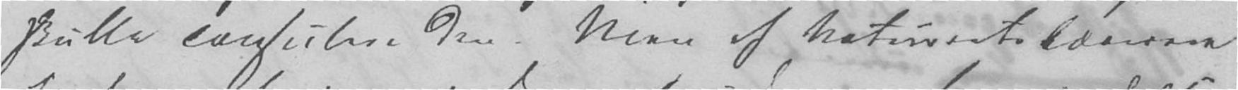skulle consultere de. Men af Naturretslærerne
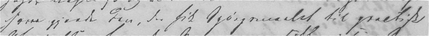som gjorde den, der fik Spørgsmaalet til practisk
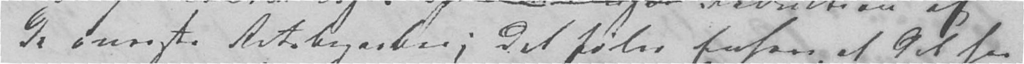de øverste Retsbegreber; det føler Enhver, at det her
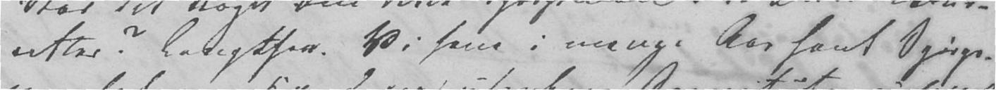retter? Lebigtsen. Vi have i mange Aar havt Spørgs-
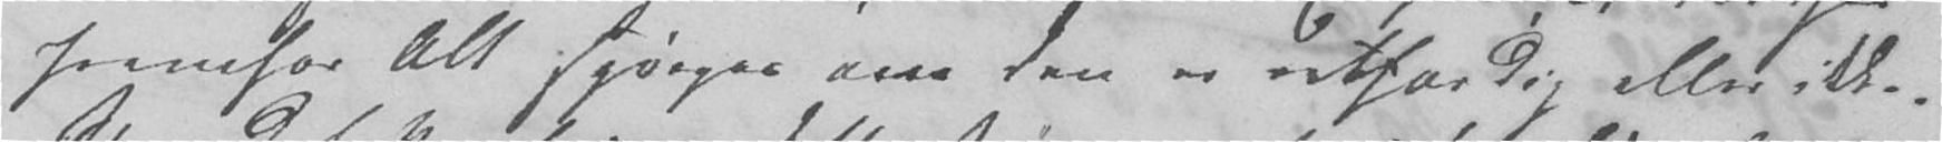fremfor Alt spørges om den er retfærdig eller ikke.
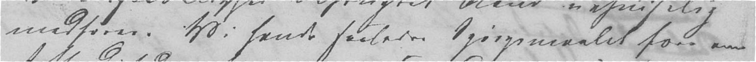medfører. Vi havde saaledes Spørgsmaalet fore om
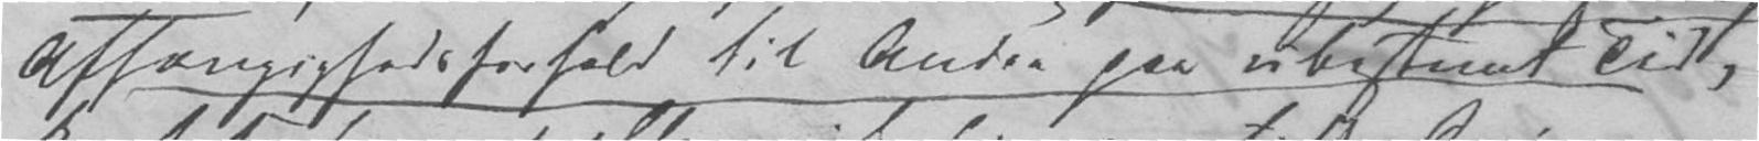Afhængighedsforhold til Andre paa ubeftemt Tid,
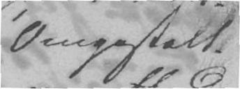Omgestalt-
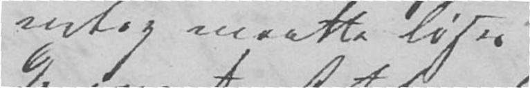netop maatte løses
